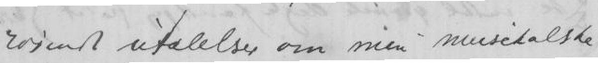rørende utalelser om min musikalske
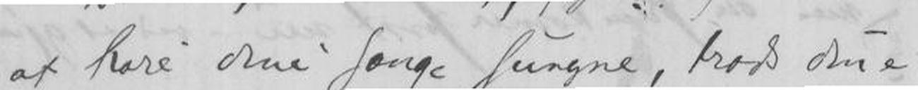at høre dine sange sungne, trods dine
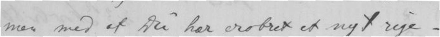men med at Du har erobret et nyt rige -
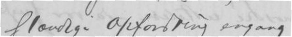stændige opfordring engang
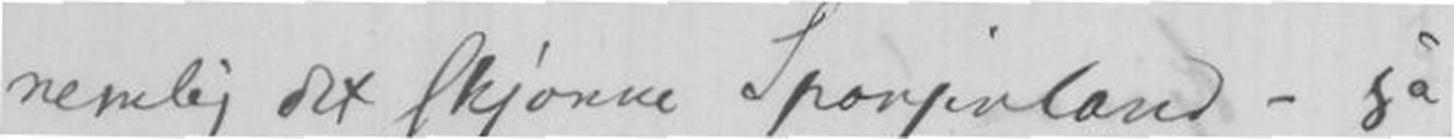nemlig det skjønne Spanjenland - så
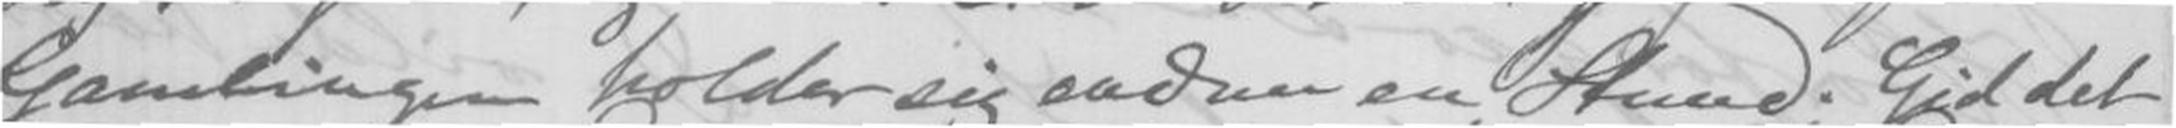Gamlingen holder sig endnu en Stund; Gid det
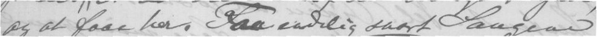og at faae her. Faa endelig snart Sangene
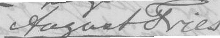August Fries
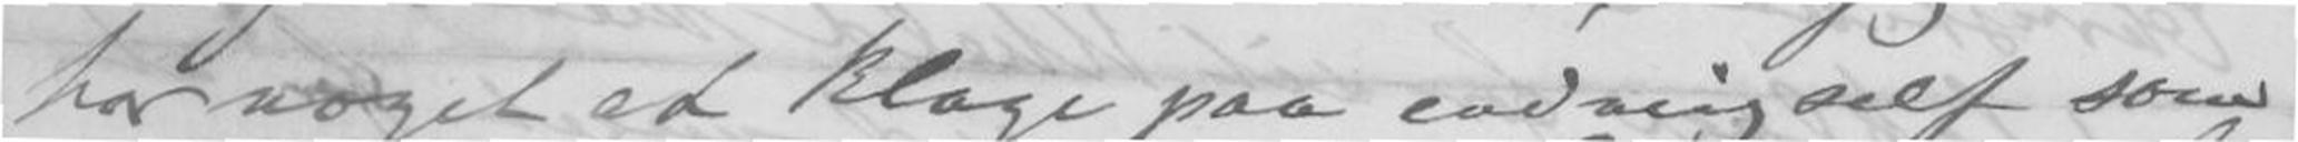har noget at klage paa end mig self som
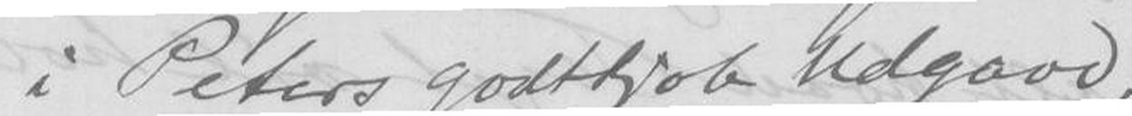i Peters godtkjøb Udgave,
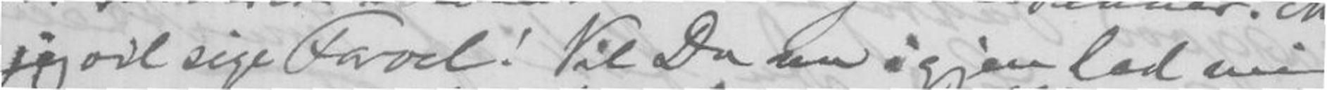jeg vil sige Farvel! Vil Du nu igjen lad mig
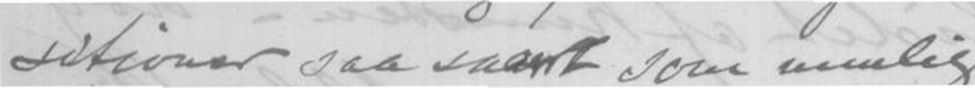sitioner saa snart som mulig
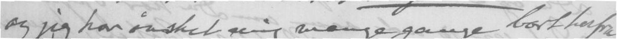og jeg har ønsket mig mange gange bort herfra.
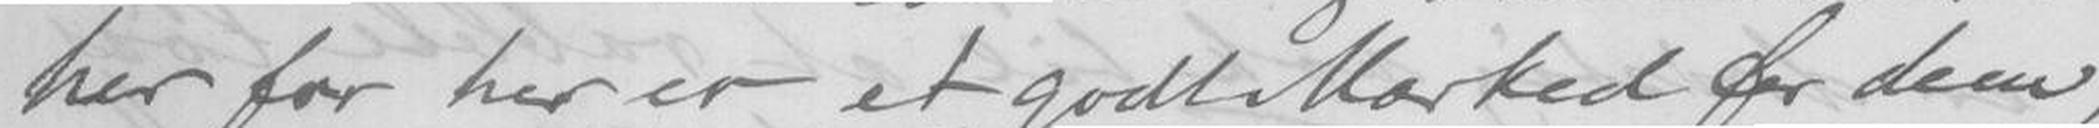her for her er et godt Market for dem,
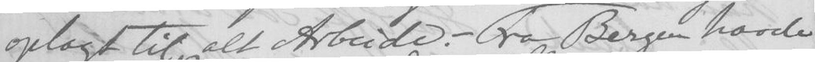oplagt til alt Arbeide. - Fra Bergen havde
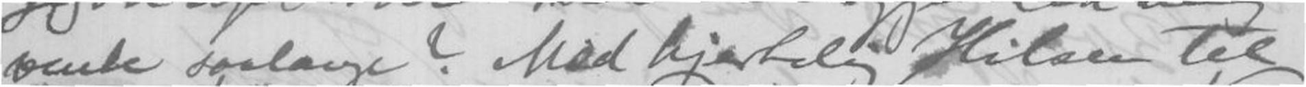vente saalenge? Med hjertelig Hilsen til
